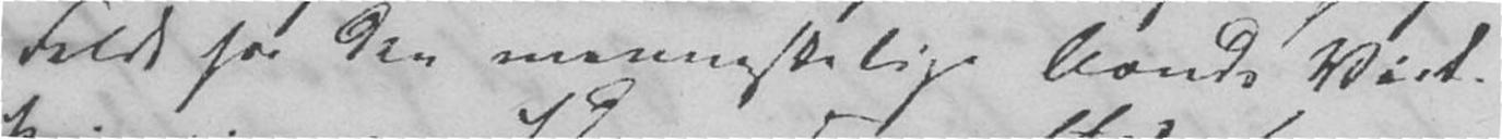Feldt for den menneskelige Aands Virk-
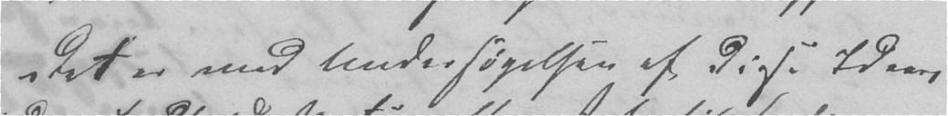Det er med Undersøgelsen af disse Ideers
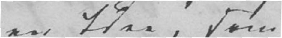en Idee,
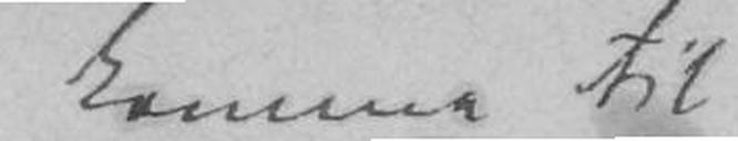komme til
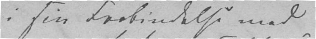i sin Forbindelse med
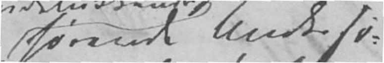hørende Undersø-
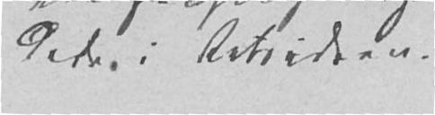dede i Retsideen.
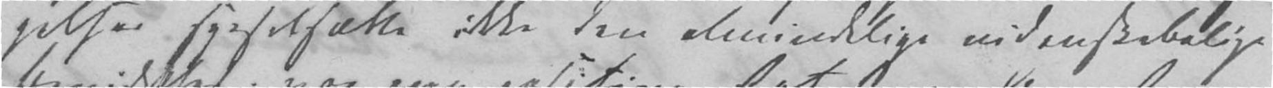gelser sysselsætte ikke den almindelige videnskabelige
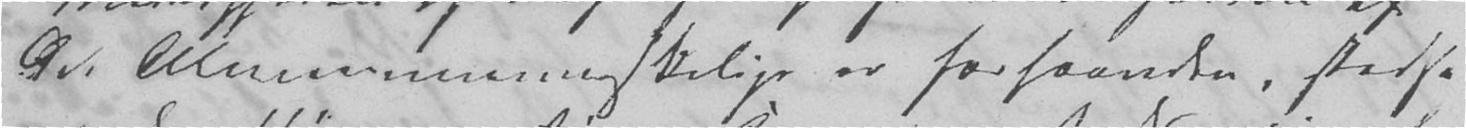det Almeenmenneskelige er forhaanden, stedse
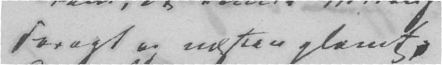Foragt og næsten glemt.
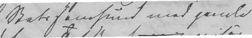Statssamfund med gamle
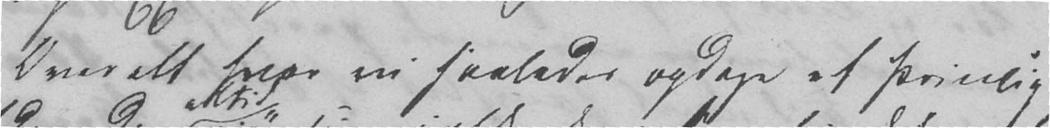Overalt hvor vi saaledes opdage et Princip
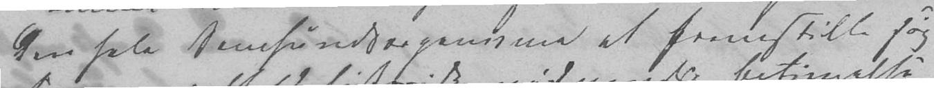den hele Samfundsorganisme at fremstille sig
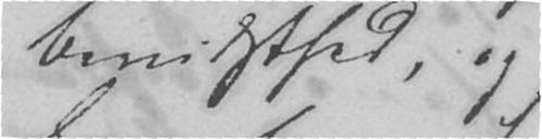Bevidsthed, og
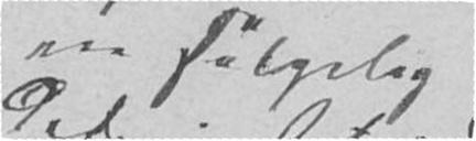ere følgelig
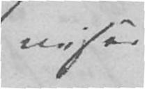viser
